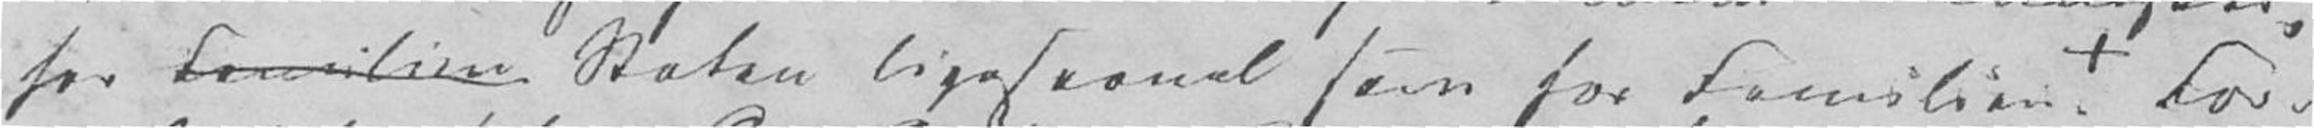for Familien Staten ligesaavel som for Familien. For-
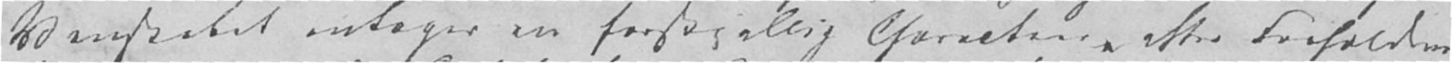Venskabet antager en forskjellig Characteer, efter Forholdenes
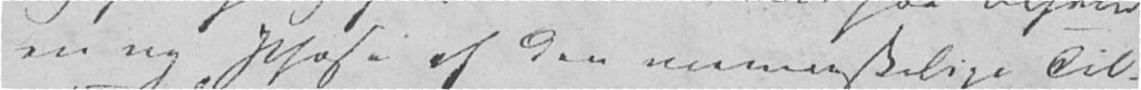en ny Phase af den menneskelige Til-
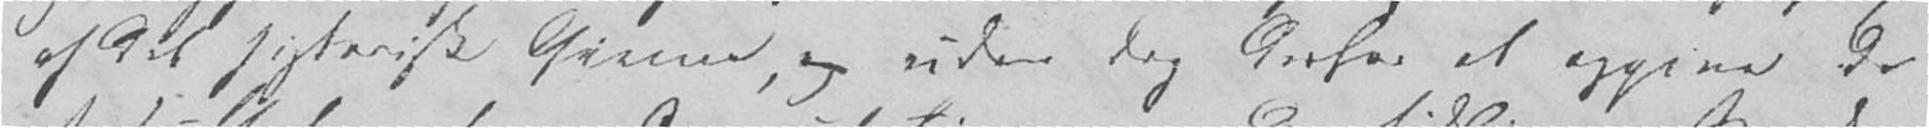af det historisk Givne, og uden dog derfor at opgive de
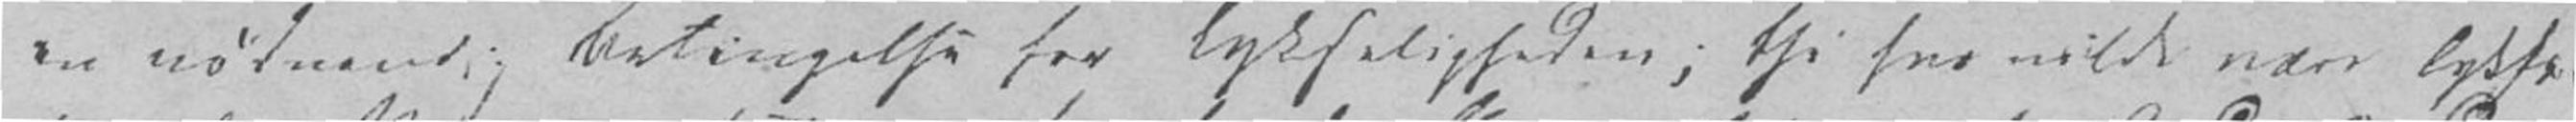en nødvendig Betingelse for Lyksaligheden; thi hvo vilde være lykke-
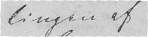lingen af
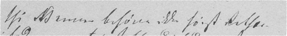thi Venner behøve ikke først Retfær-
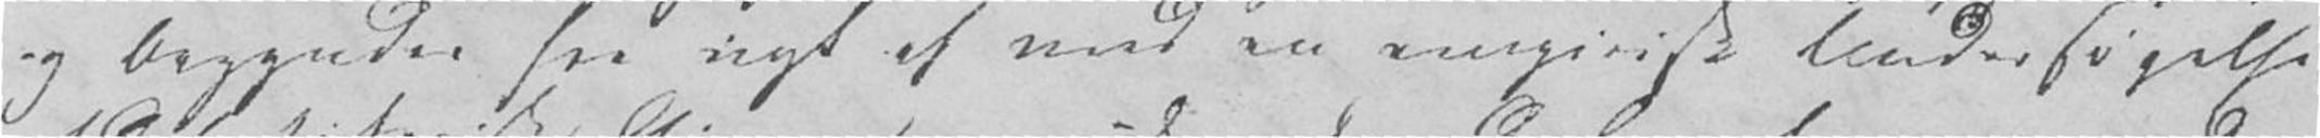og begynder fra nyt af med en empirisk Undersøgelse
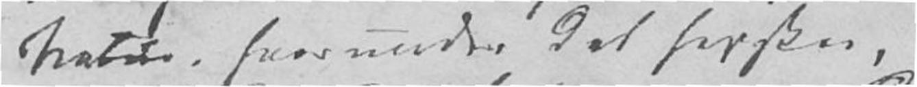Natur, hvorunder det hersker,
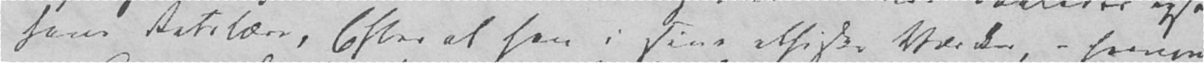i hans Retslære, Efter at han i sine etiske Værker, - fornem-
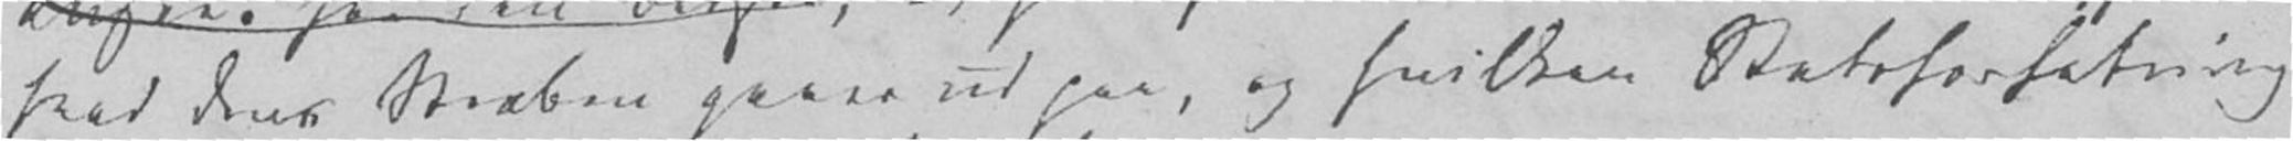hvad dens Stræben gaaer ud paa, og hvilken Statsforfatning
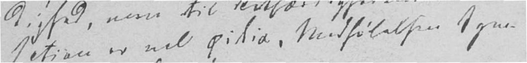sation er vel gilid, Medfølelsen Sym-
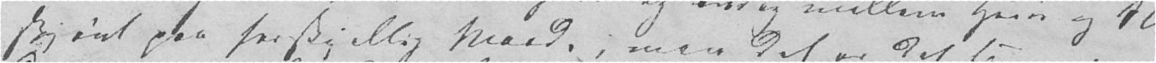skjønt paa forskjellig Maade; men det er det, som giver
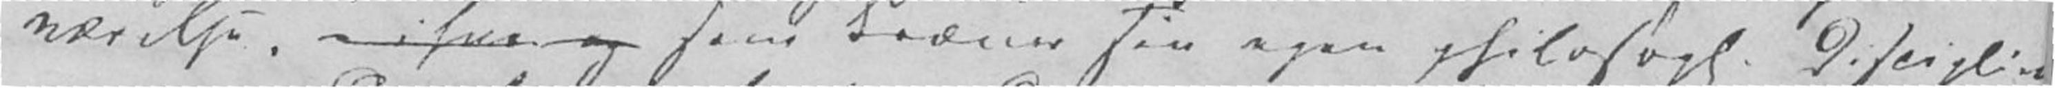værelse, - ifra og som kræver sin egen philosoph. Disciplin
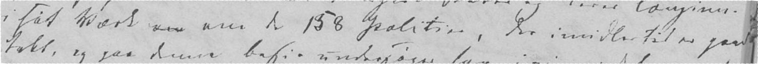i sit Værk om om de 158 Politier, der imidlertid er gaat
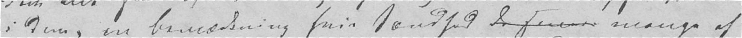i dem, en Bemærkning hvis Sandhed de senere mange af
